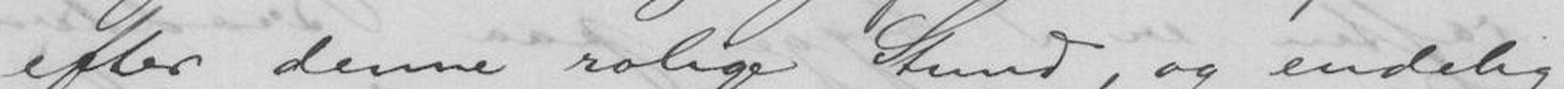efter denne rolige Stund, og endelig
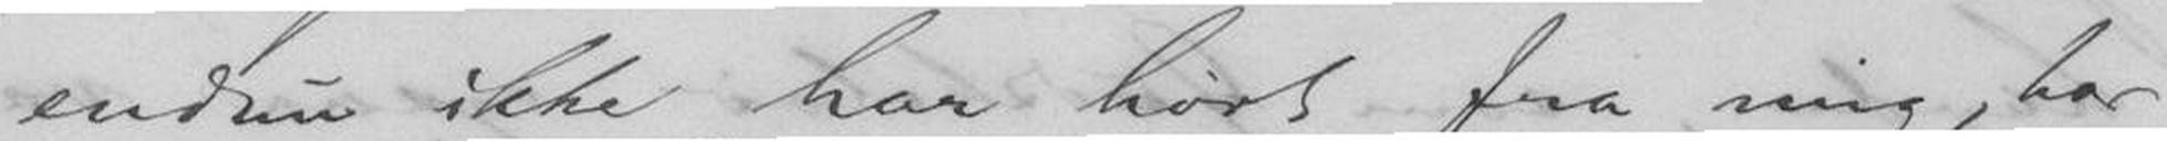endnu ikke har hørt fra mig, har
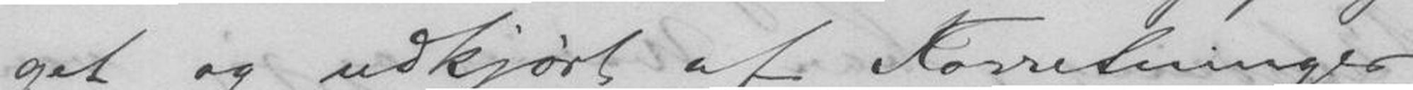get og udkjørt af Forretninger
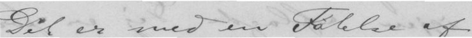Det er med en Følelse af
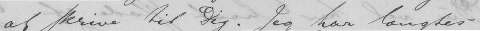at skrive til Dig. Jeg har længtes
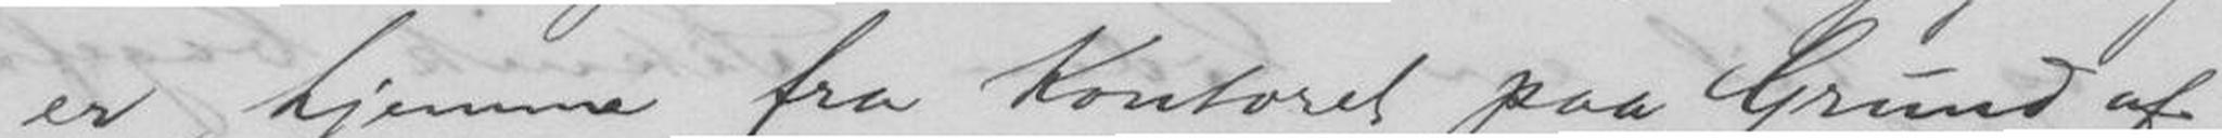er hjemme fra Kontoret paa Grund af
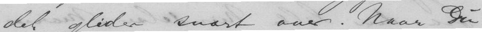det glider snart over. Naar Du
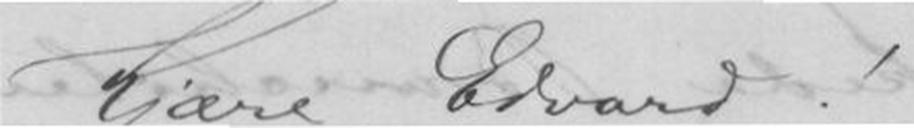Kjære Edvard!
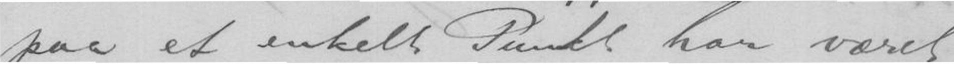paa et enkelt Punkt har været
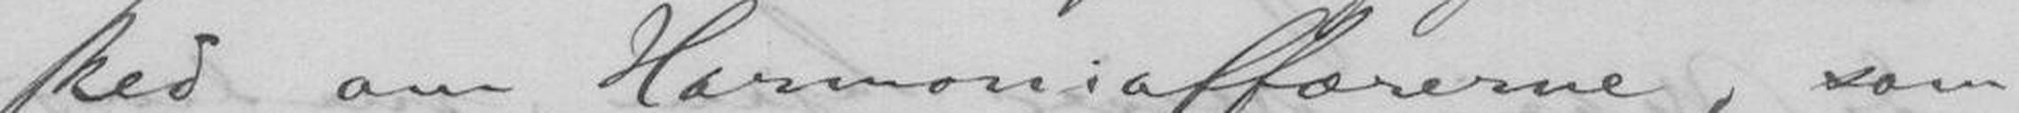sked om Harmoniaffærerne, som
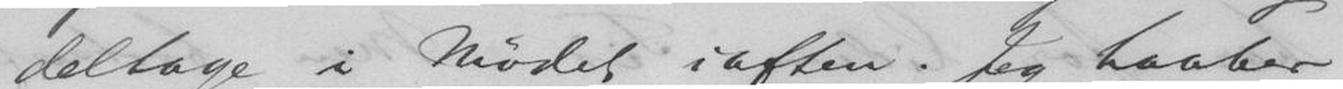deltage i Mødet iaften. Jeg haaber,
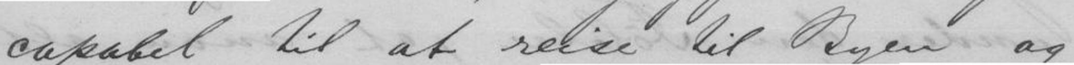capabel til at reise til Byen og
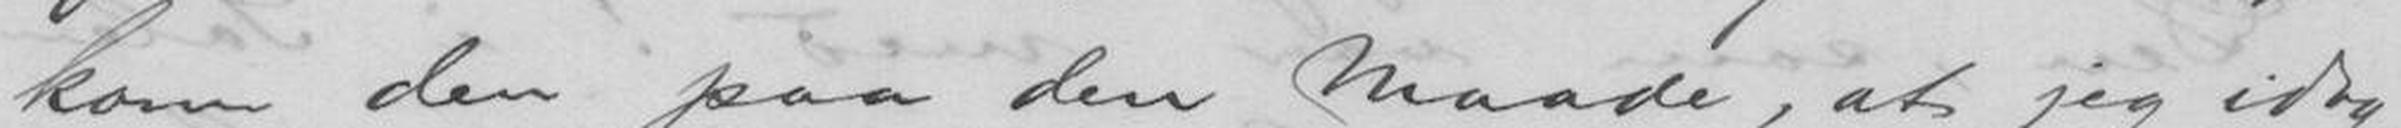kom den paa den Maade, at jeg idag
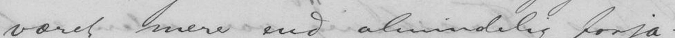været mere end almindelig forja-
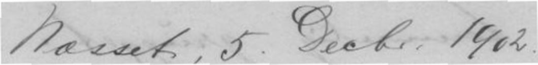Næsset, 5 Decbr. 1902
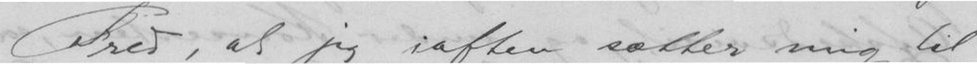Fred, at jeg iaften sætter mig til
